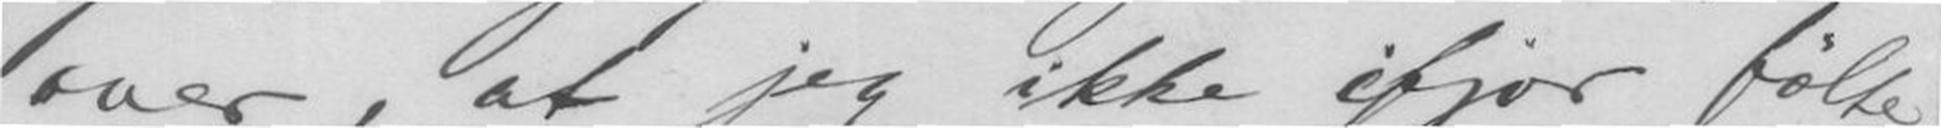over, at jeg ikke ifjor følte
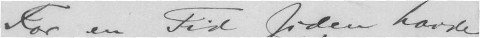For en Tid siden havde
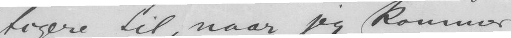tigere til, naar jeg kommer
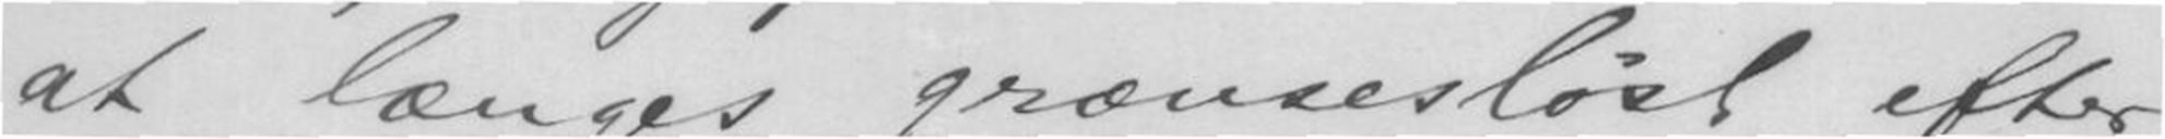at længes grænsesløst efter
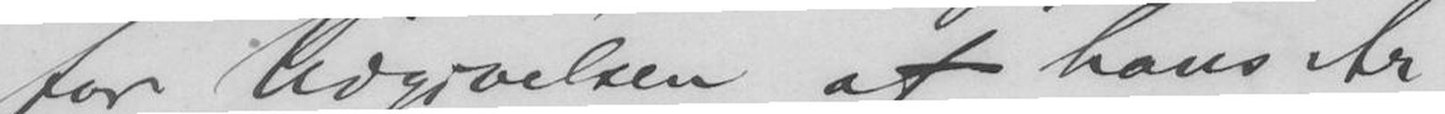for Udgivelsen af hans Ar-
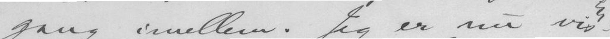gang imellem. Jeg er nu vis
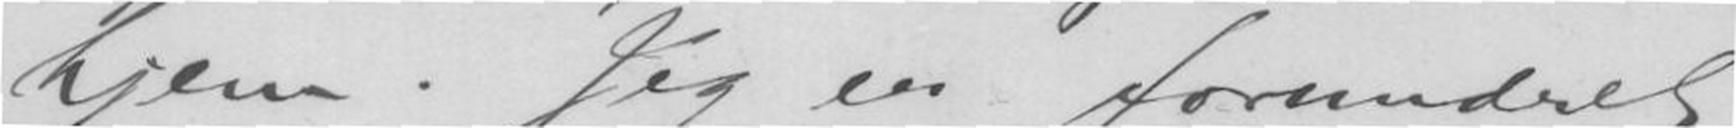hjem. Jeg er forundret
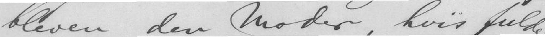bleven den Moder, hvis fulde
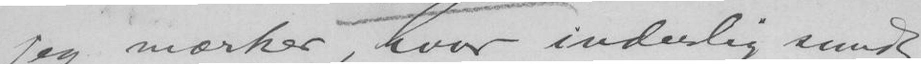jeg mærker, hvor inderlig sundt
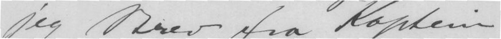jeg Brev fra Kaptein
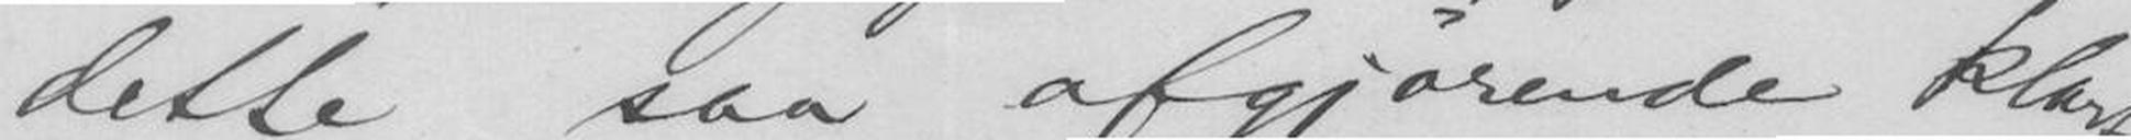dette saa afgjørende klart
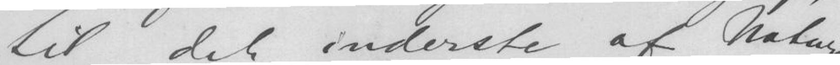til det inderste af Naturen
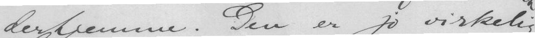derhjemme. Den er jo virkelig
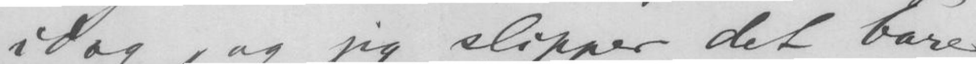idag, og jeg slipper det bare
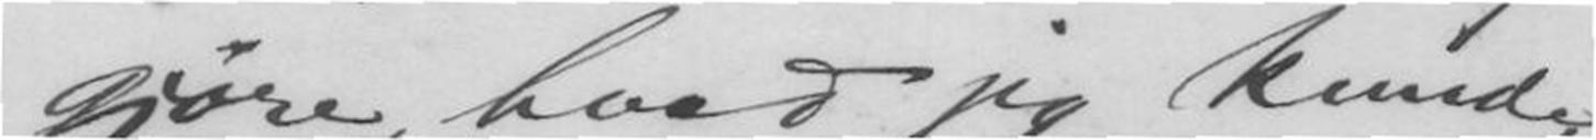gjøre, hvad jeg kunde
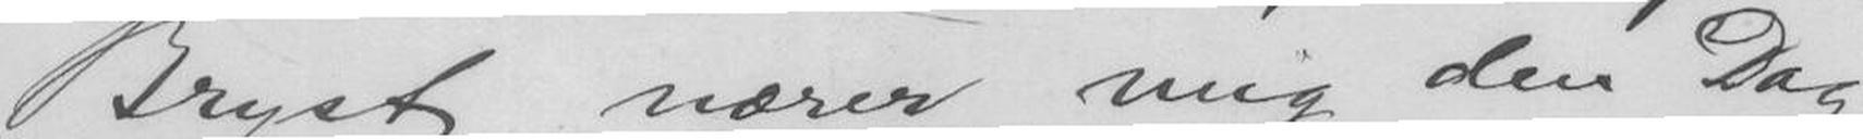Bryst nærer mig den Dag
